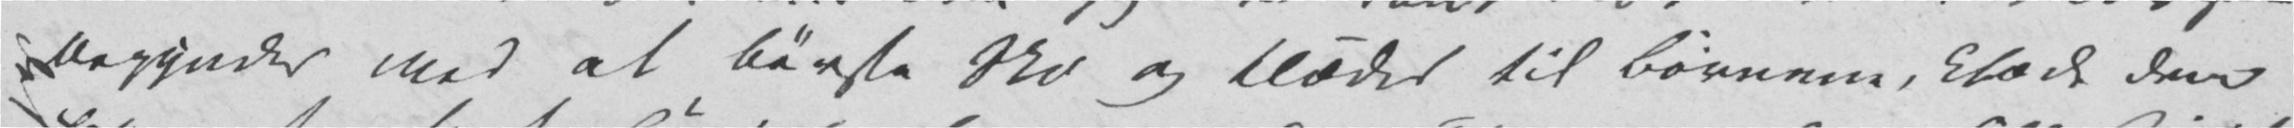begynder med at børste Sko og Klæder til Børnene, klæde dem
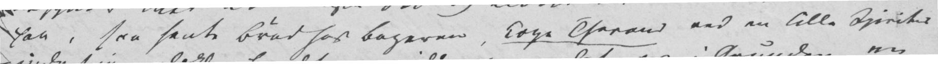paa, saa hente Brød hos Bageren, koge Thevand ved en lille Spiritus
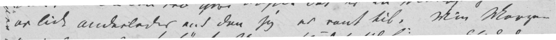er lidt anderledes end den jeg er vant til, Min Morgen
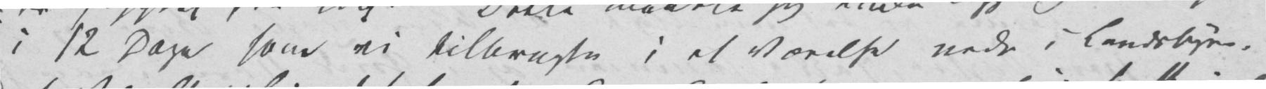i 12 Dage som vi tilbragte i et Værelse nede i Landsbyen,
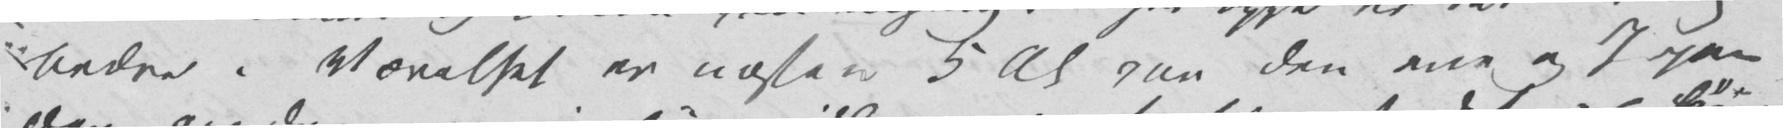bedre. Værelset er næsten 5 Al paa den ene og 7 paa
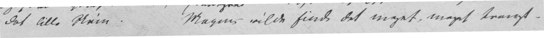det lille Skrin. Magen – vilde finde det meget, meget trangt.
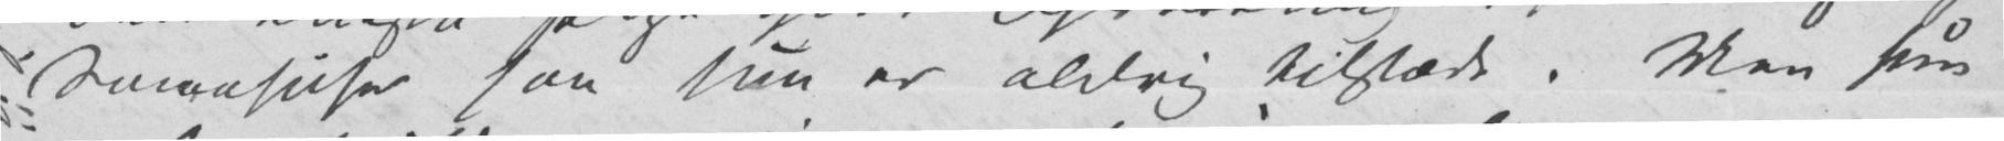Smaahuse saa hun er aldrig tilstæde. Men hun
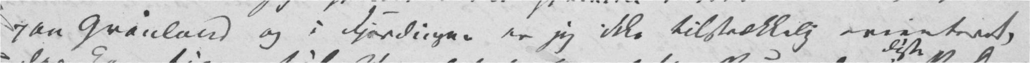paa Grønland og i Fjerdingen er jeg ikke tilstrækkelig orienteret,
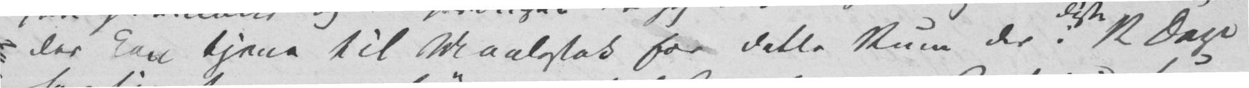der kan tjene til Maalestok for dette Rum der i 12 Dage
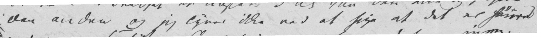den anden og jeg lyver ikke ved at sige at det er høiere
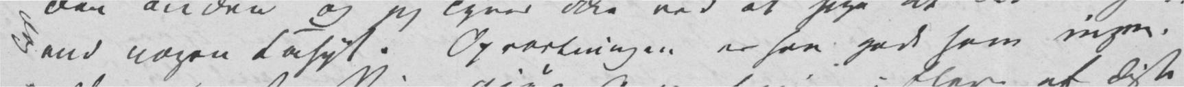end nogen Kahyt. Opvartningen er saa godt som ingen.
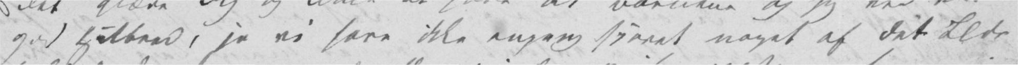god Helbred, ja vi have ikke engang sporet noget af det Ilde
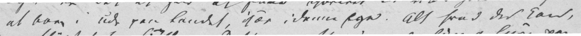at boe i ude paa Landet, især i denne Egn. Alt hvad der kan,
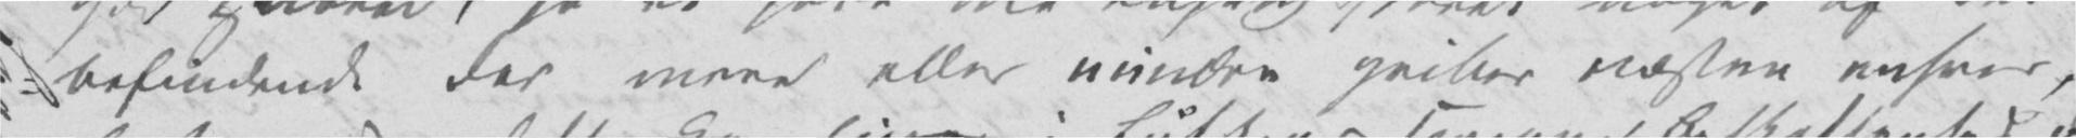befindende der mere eller mindre griber næsten enhver,
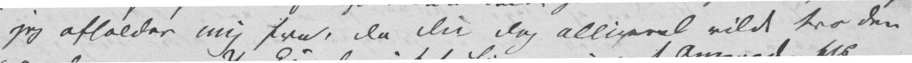jeg afholder mig fra, da Du dog alligevel vilde tro den
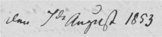Den 7de August 1853
